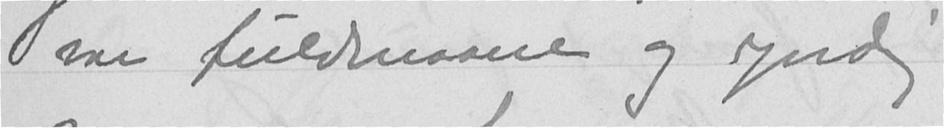var fuldmaane og yndig
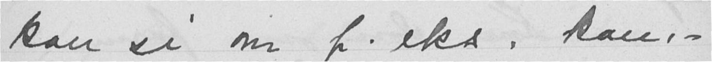kan si om f.eks. kam-
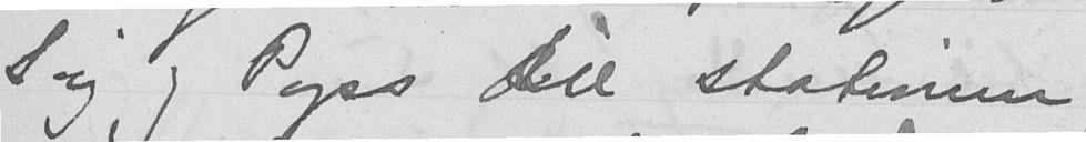Sig og Paps til stationen
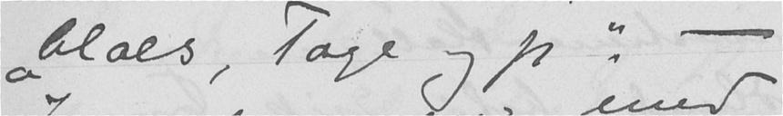"Claes, Tage og jeg." -
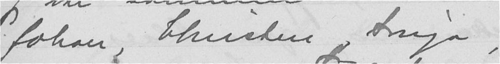Johan, Christen, Sonja,
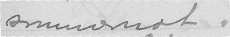sommernat.
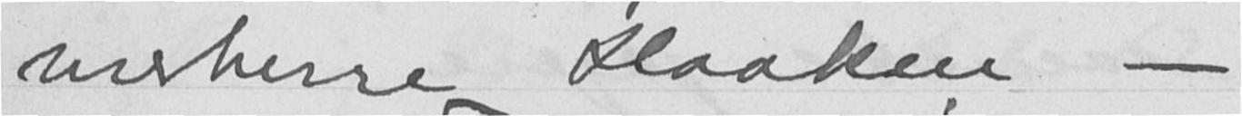merherre Haaken -
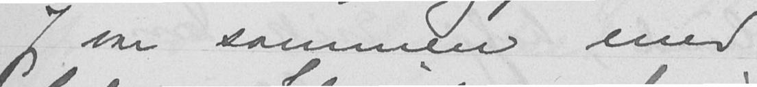Jeg var sammen med
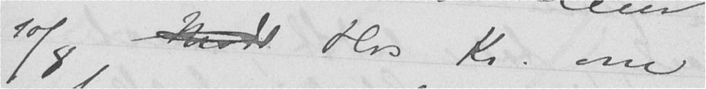10/8 Midd Hos Kr. om
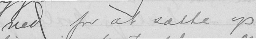ned for at sætte op
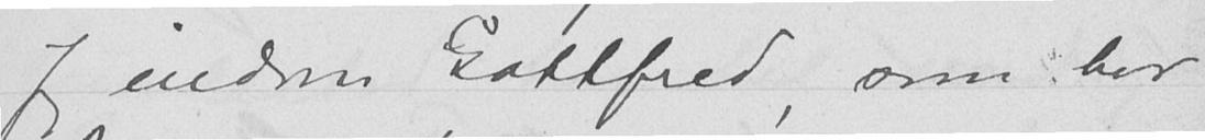Jeg indom Gottfred, som har
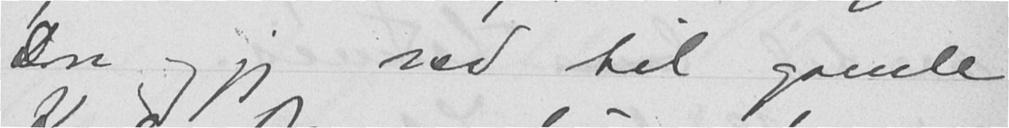Don og jeg ned til gamle
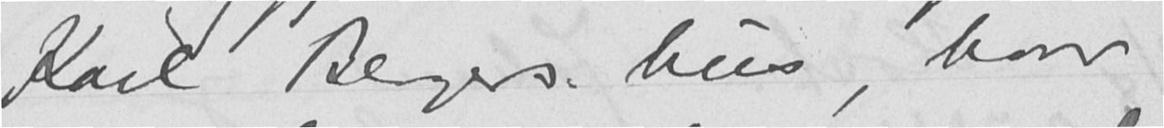Karl Bergers hus, hvor
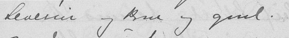Severin og kone og gaml.
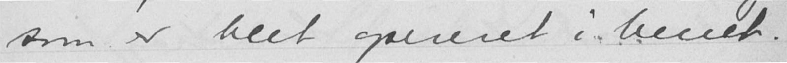som er blit opereret i benet.
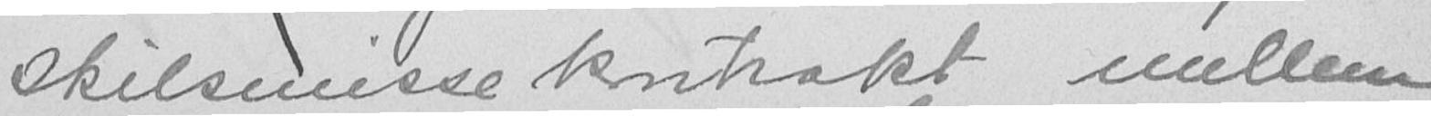skilsmissekontrakt mellem
